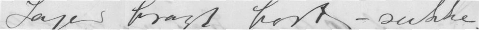Sager bragt bort - sukke
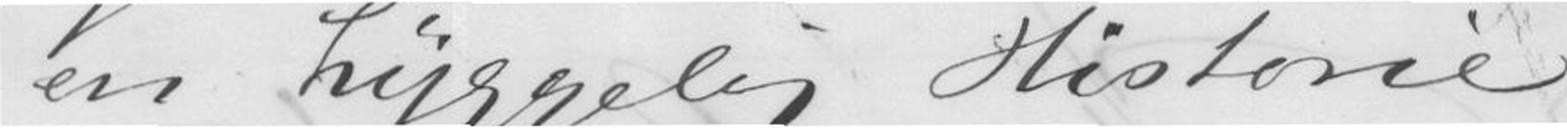en hyggelig Historie
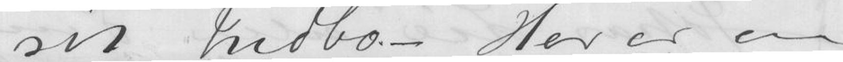sit Indbo. - Her er en
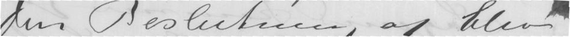Din Beslutning at blive
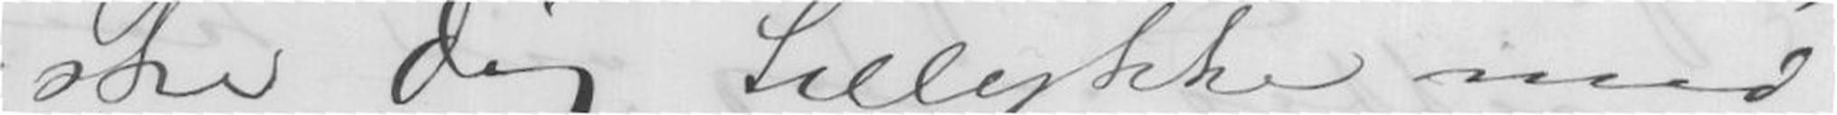ske Dig tillykke med
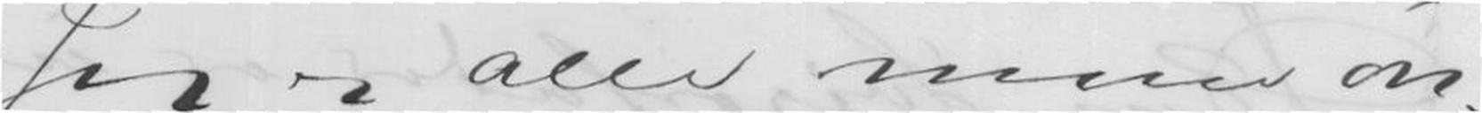Jeg og alle mine øn
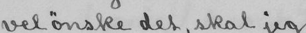vel ønske det, skal jeg
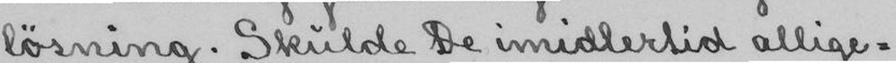løsning. Skulde De imidlertid allige-
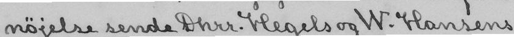nøjelse sende Dhrr. Hegels og W. Hansens
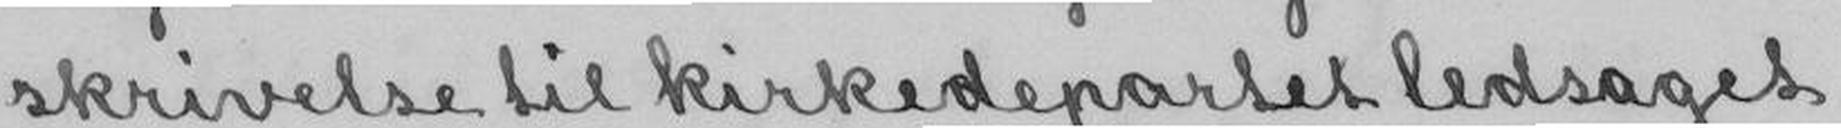skrivelse til kirkedepartet ledsaget
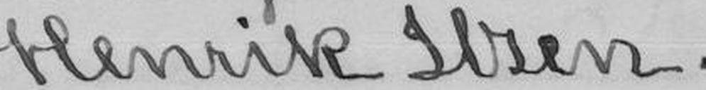Henrik Ibsen.
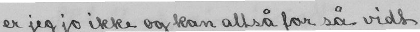er jeg jo ikke og kan altså for så vidt
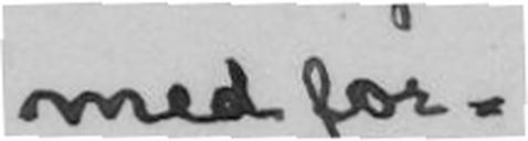med for-
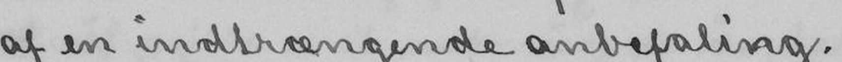af en indtrængende anbefaling.
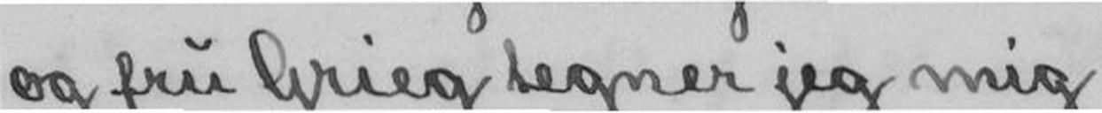og fru Grieg tegner mig
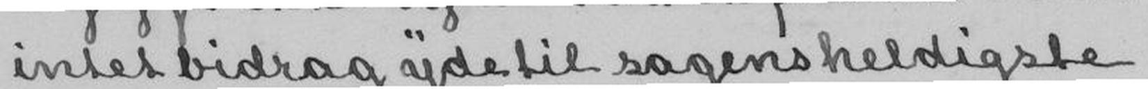intet bidrag yde til sagens heldigste
